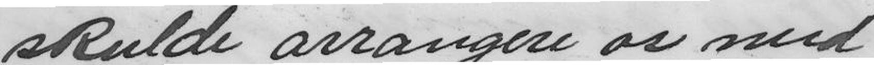skulde arrangere os med
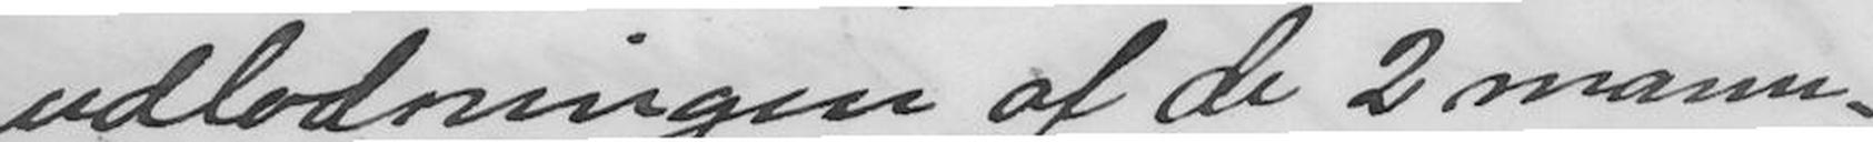udlodningen af de 2 manu-
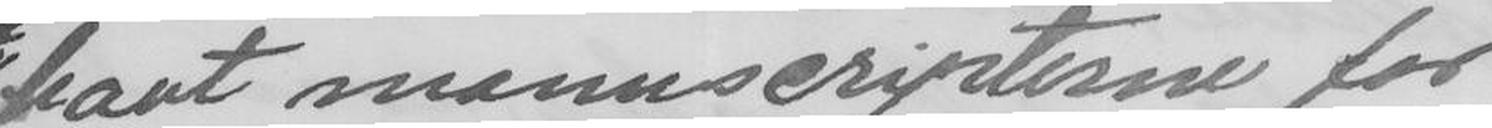havt manuscripterne for
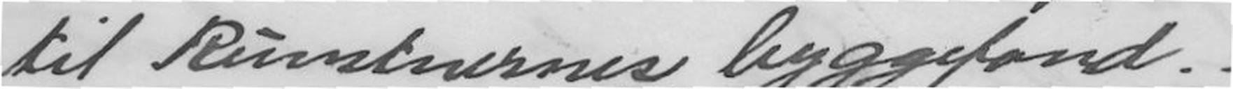til kunstnernes byggefond.
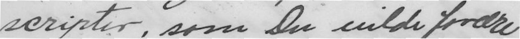scripter, som Du vilde forære
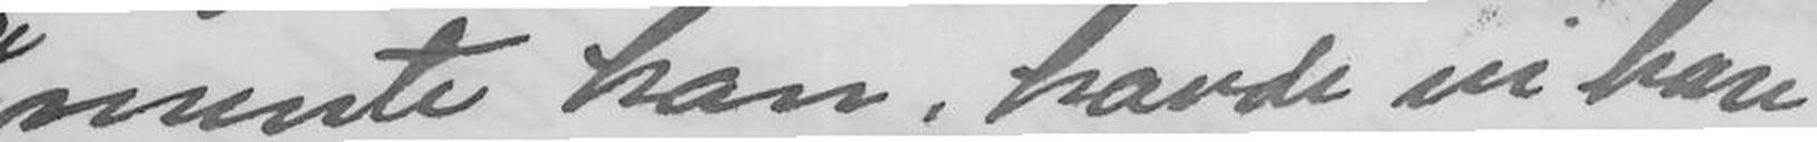mente han, havde vi bare
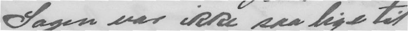Sagen var ikke saa lige til
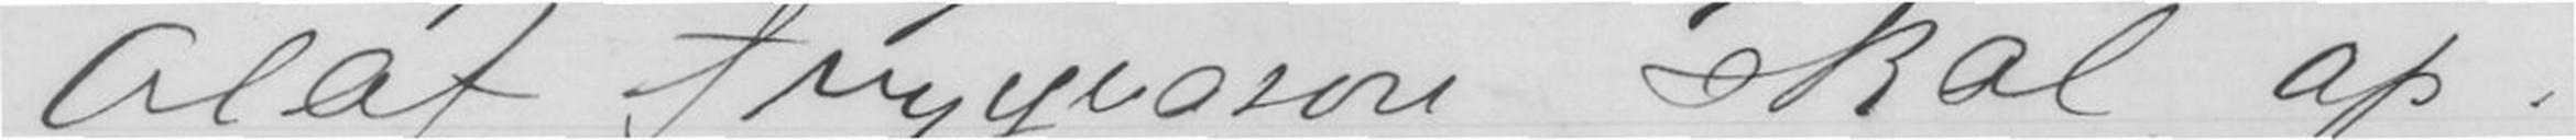Olaf Trygvason skal op!
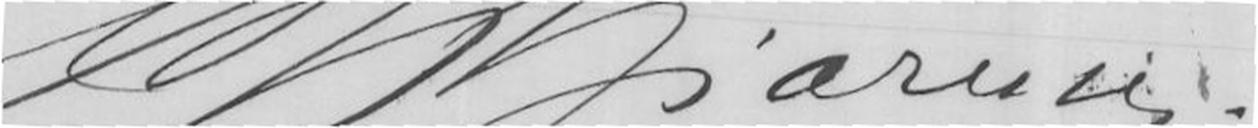B Bjørnson
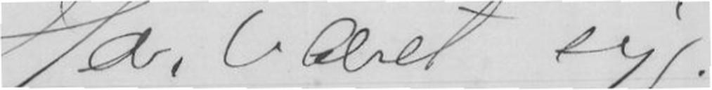Har været syg!
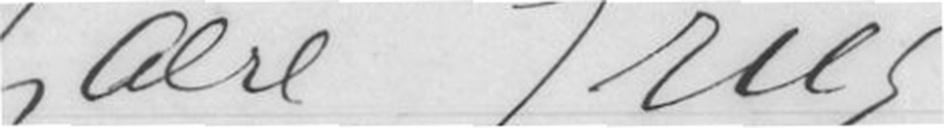Kjære Grieg
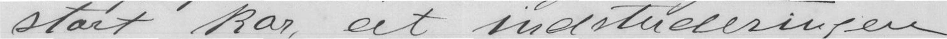stort kor, at indstuderingen
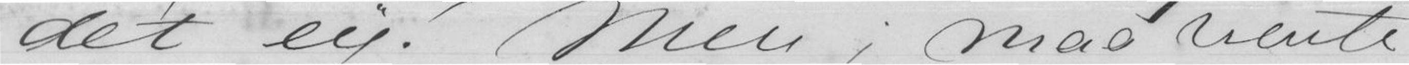det ey! Men jeg maa vente
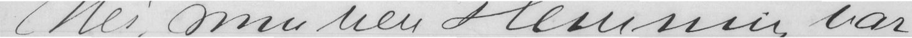Nei min ven stemning var
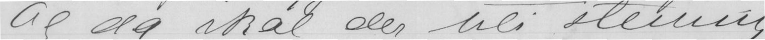Og da skal der bli stemning.
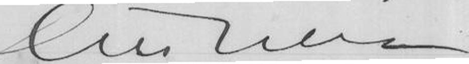Din heng
